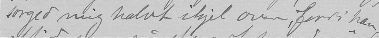sorged mig halvt ihjel over, fordi han
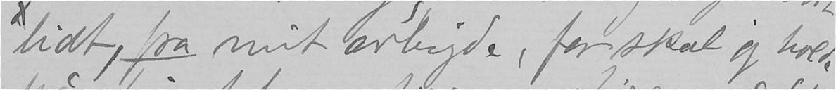lidt, fra mit arbejde, for skal jeg holde
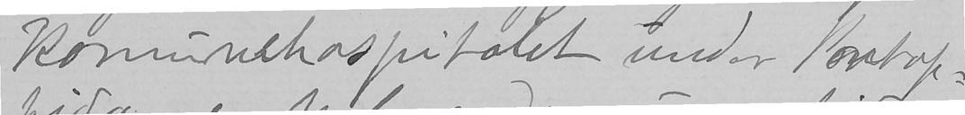komunehospitalet under Pontop-
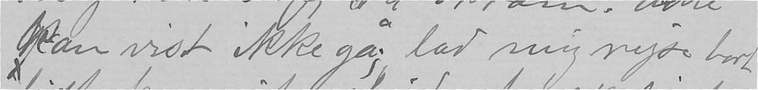kan vist ikke gå; lad mig rejse bort
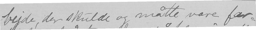bejde, der skulde og måtte være fær-
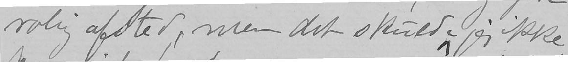rolig afsted, men det skulde jeg ikke
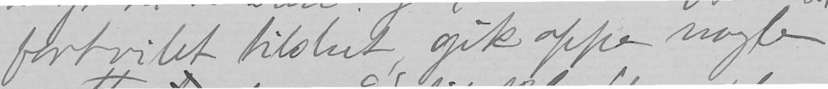fortvilet tilslut, gik oppe nogle
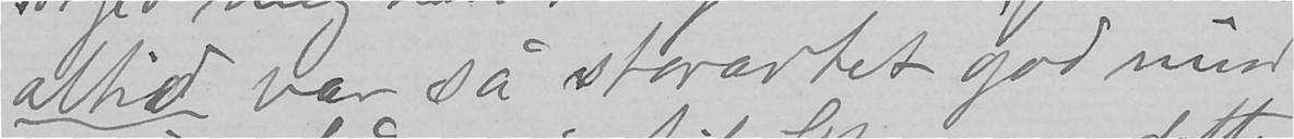altid var så storartet god med
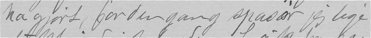ha gjort, for dengang spasær jeg lige
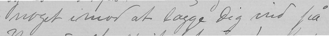noget imod at lægge Dig ind på
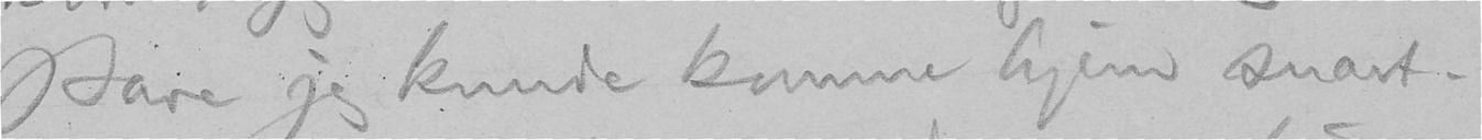Bare jeg kunde komme hjem snart.
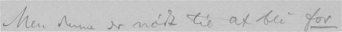Men denne er nødt til at bli for
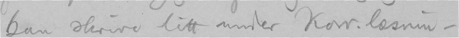han skrive litt under Korr.læsnin-
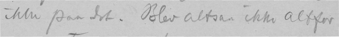ikke paa det. Blev altsaa ikke altfor
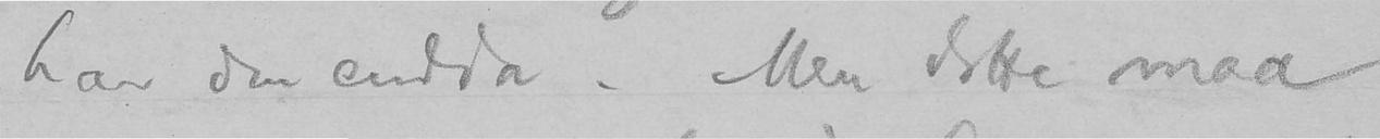har den endda. Men dette maa
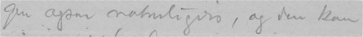gen ogsaa naturligvis, og den kan
